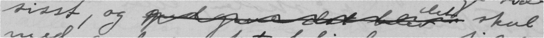sisst, og  skal
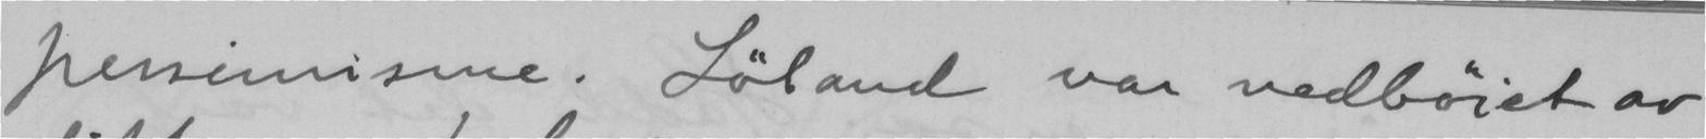pessimisme. Løland var nedbøiet av
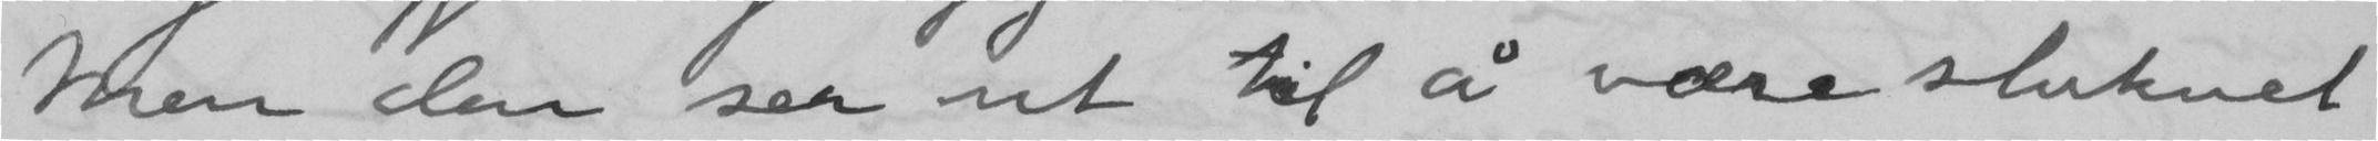Men den ser ut til å være sluknet
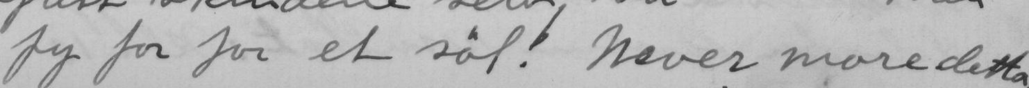jeg for for et søl! Never more detta,
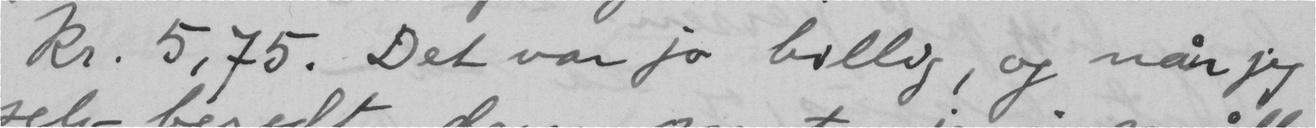kr. 5, 75. Det var jo billig, og når jeg
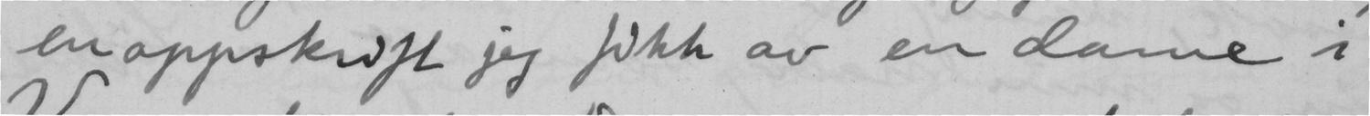en oppskrift jeg fikk av en dame i
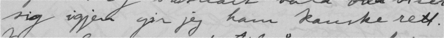sig igjen gir jeg ham kanske rett.
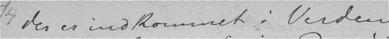der er indkommet i Verden.
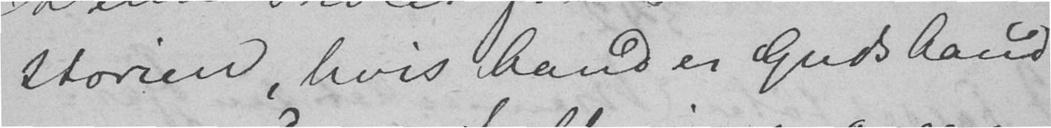storien, hvis Aand er Guds Aand
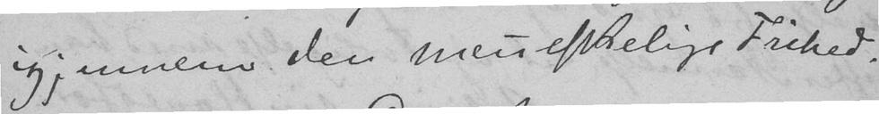igjennem den menneskelige Frihed.
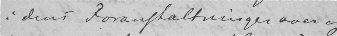i dens Foranstaltninger over og
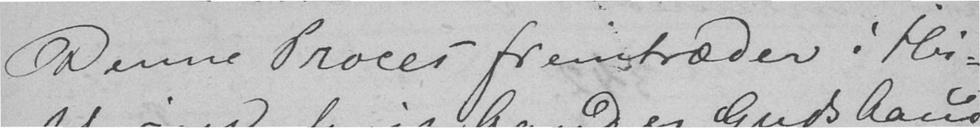Denne Proces fremtræder i Hi-
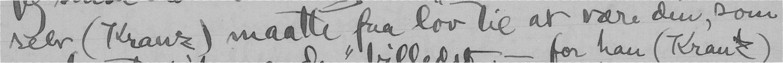selv (Kranz) maatte "faa lov" til at være den, som
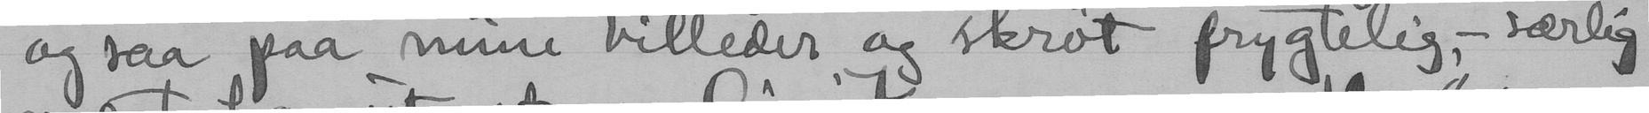og saa paa mine billeder og skrøt frygtelig, - særlig
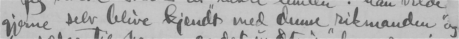gjerne selv blive kjendt med denne "rikmanden" og
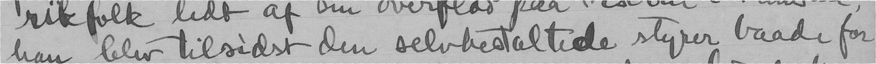han blev tilsidst den selvbestaltede styrer baade for
